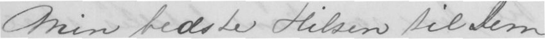Min bedste Hilsen til Dem
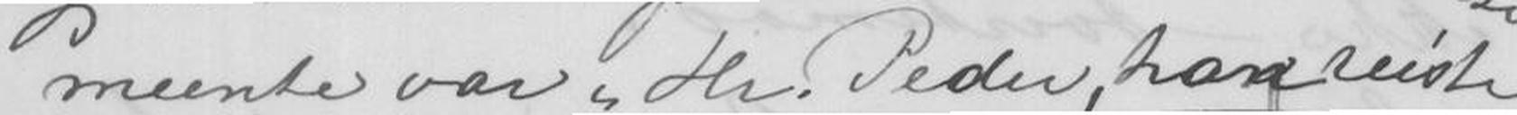jeg meente var Hr. Peder, han reiste
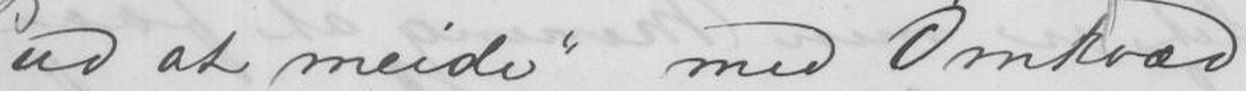ud at meide med Omkvæd
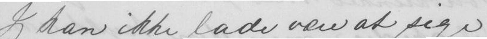Jeg kan ikke lade være at sige
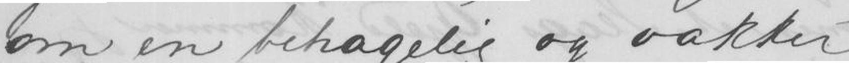om en behagelig og vakker
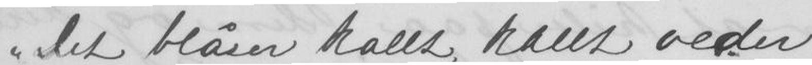det blåser kallt, kallt veder
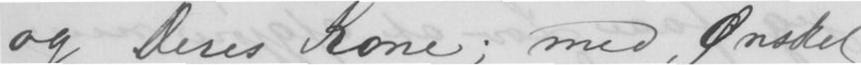og Deres Kone; med Ønsket
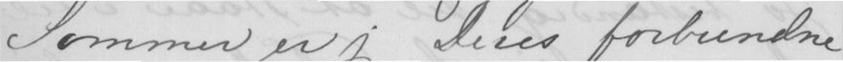Sommer er jeg Deres forbundne
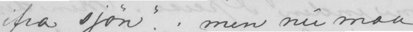ifra sjøn; men nu maa
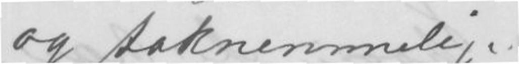og taknemmelig
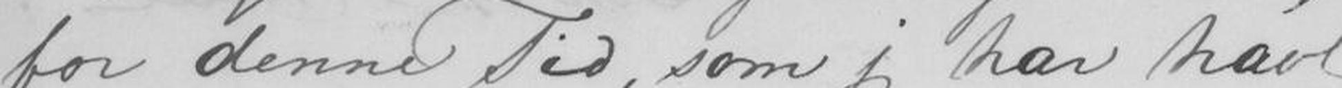for denne Tid, som jeg har havt
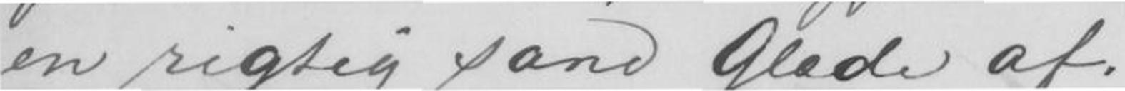en rigtig sand Glæde af.
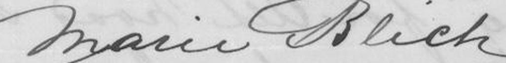Marie Blich
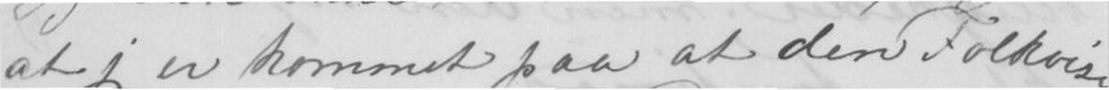at jeg er kommet paa at den Folkevise
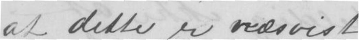at dette er næsvist.
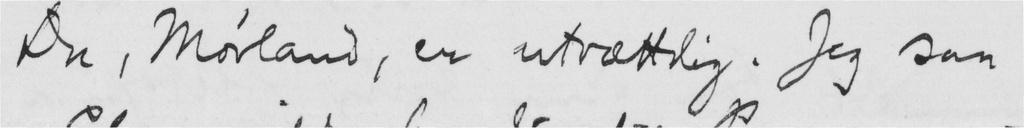Du, Mørland, er utrættelig. Jeg saa
